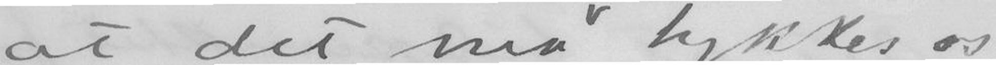at det nå lykkes os
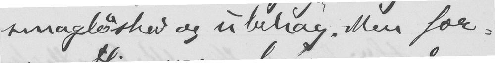smagløshed og ubehag. Men for-
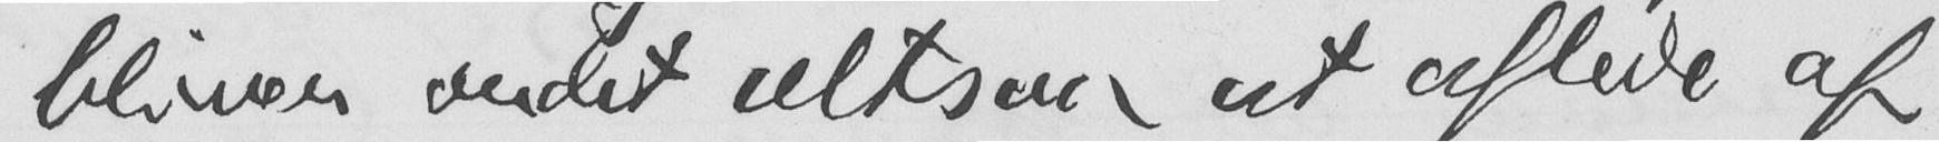bliver ordet altsaa at aflede af
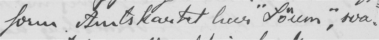form. Amtskartet har "Søum", sva-
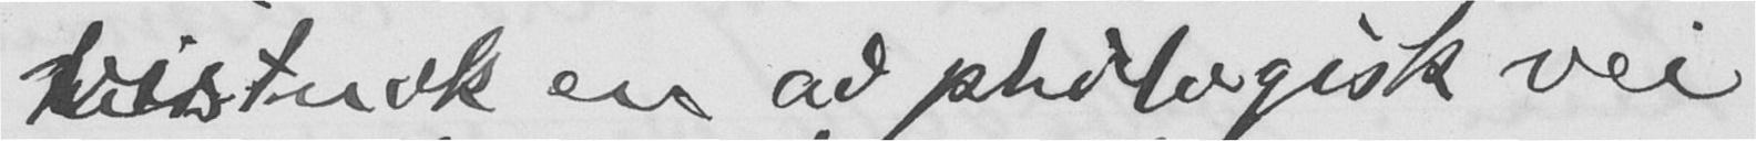visstnok en ad philologisk vei
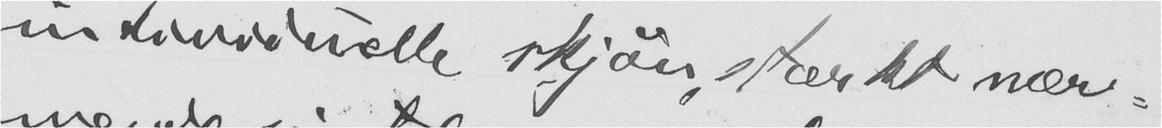individuelle skjøn, stærkt nær-
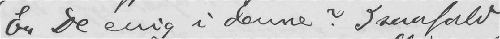Er De enig i denne? I saafald
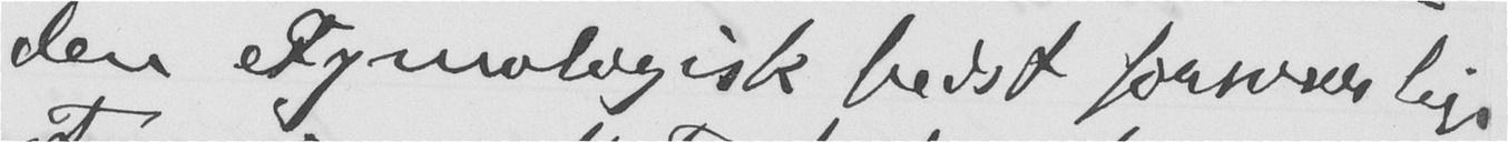den etymologisk bedst forsvarlige
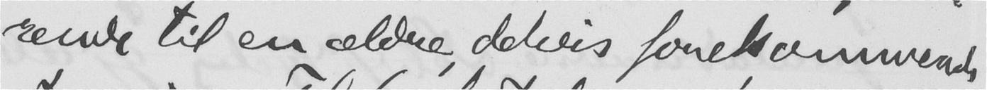rende til en ældre delvis forekommende
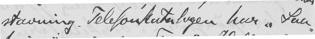stavning. Telefonkatalogen har "Saa-
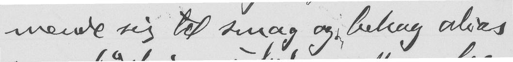mende sig til smag og behag alias
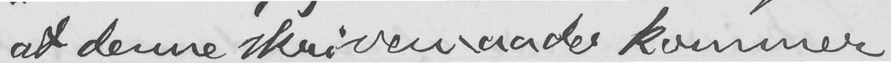at denne skrivemaade kommer
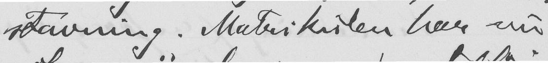stavning. Matrikulen har nu
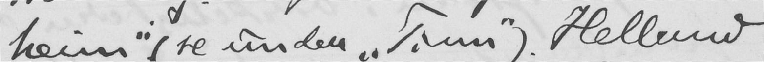heim" (se under "Tinn"). Helland
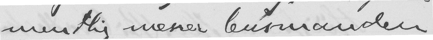mentlig mener lensmanden
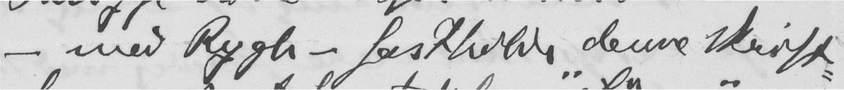- med Rygh - fastholde denne skrift-
